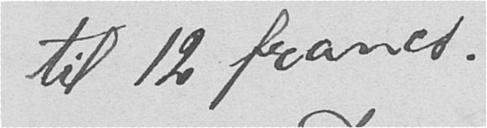til 12 francs.
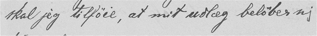skal jeg tilføie at mit udlæg beløber sig
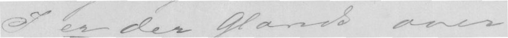I er der Glands over
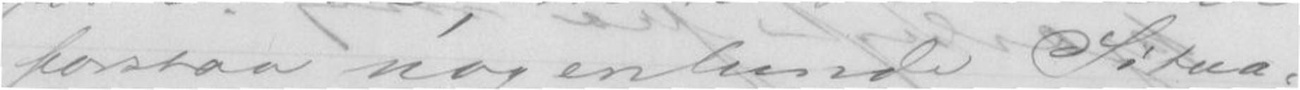forstaa nogenlunde Situa-
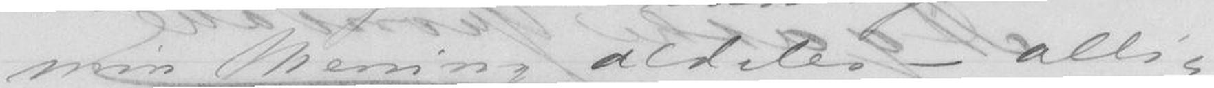min Mening aldeles - alli-
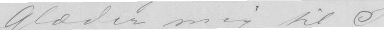Glæder mig til I
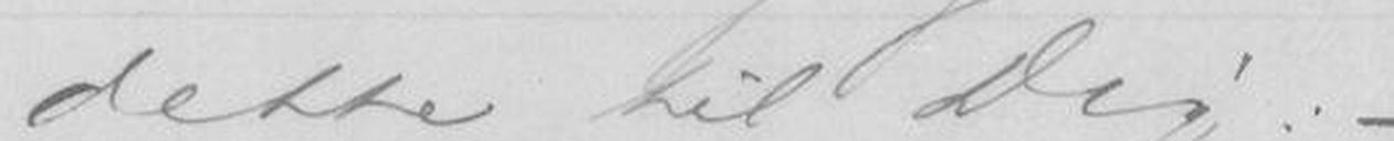dette til Dig.
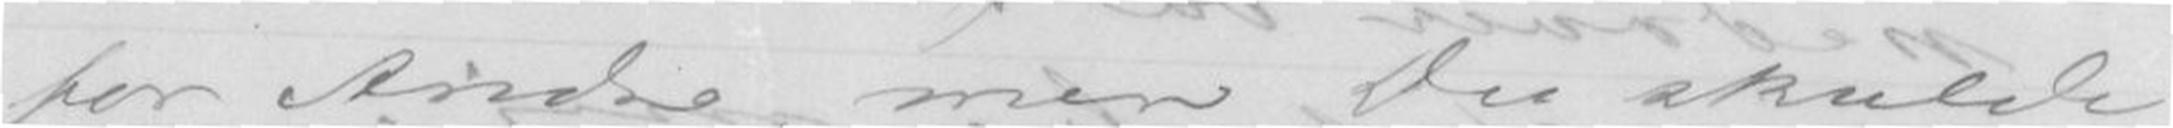for Andre, men Du skulde
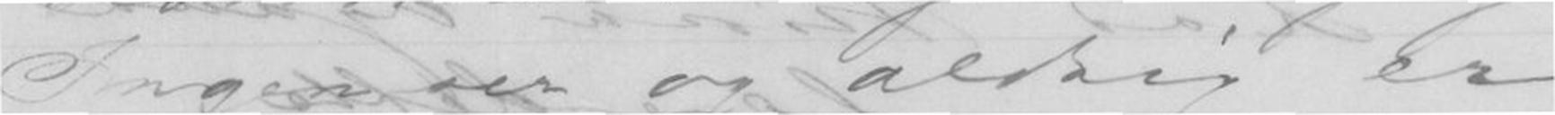Ingen ser og aldrig er
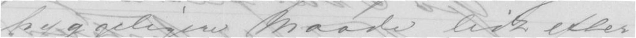hyggeligere Maade lidt efter
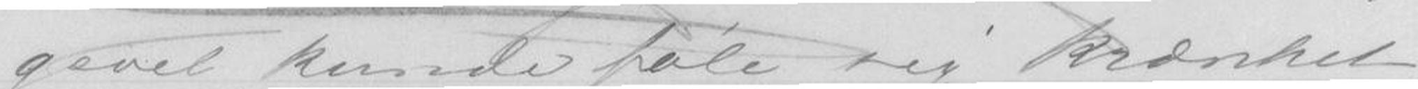gevel kunde føle sig krænket
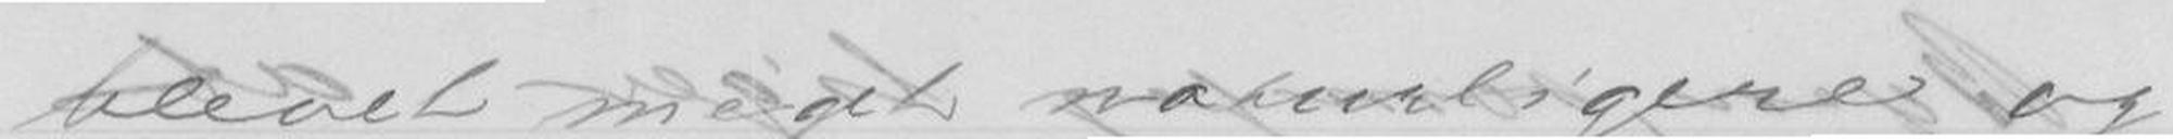blevet meget naturligere og
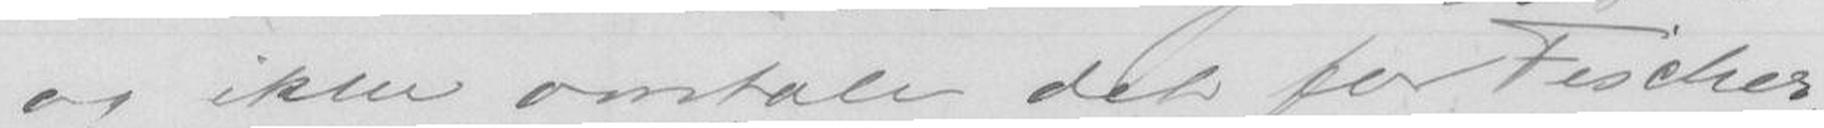og ikke omtale det for Fischer,
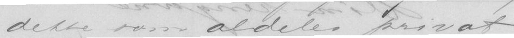dette som aldeles privat
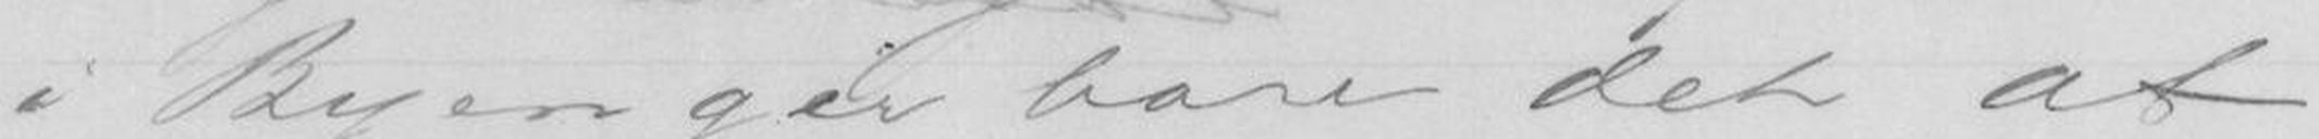i Byen gir bare det at
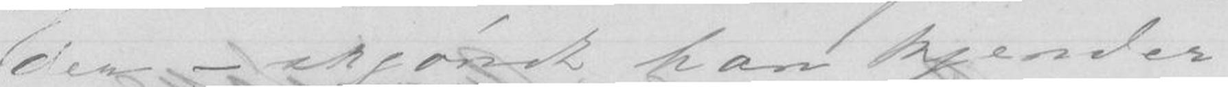der - skjøndt han kjender
